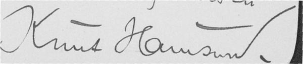Knut Hamsun.
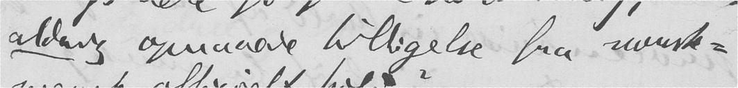aldrig opnaaede billigelse fra norsk-
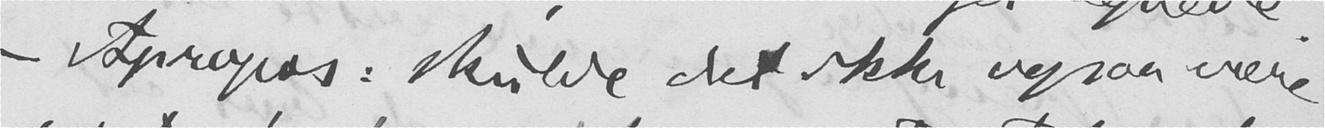- Apropos: skulde det ikke ogsaa være
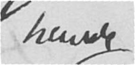havde
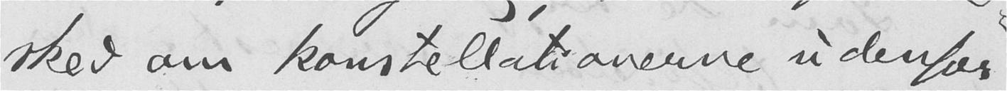sked om konstellationerne udenfor
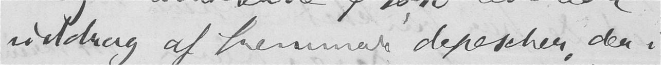uddrag af fremmede depescher, der i
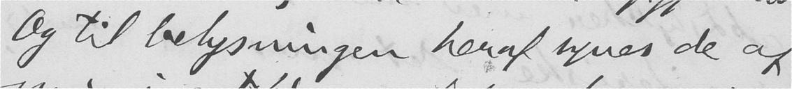Og til belysningen heraf synes de af
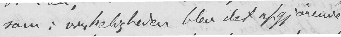som i virkeligheden blev det afgjørende.
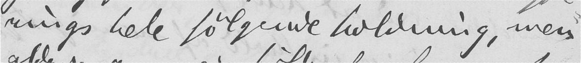rings hele følgende holdning, men
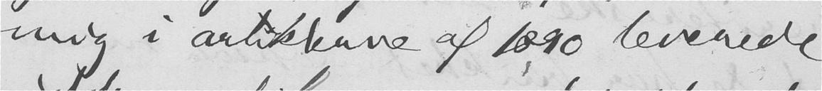mig i artiklerne af 1890 leverede
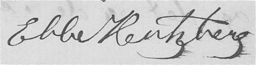Ebbe Hertzberg.
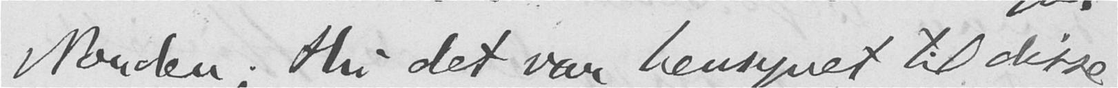Norden; thi det var hensynet til disse
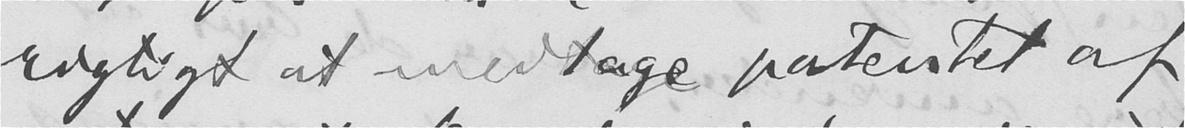rigtigt at medtage patentet af
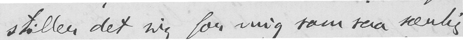stiller det sig for mig som saa særlig
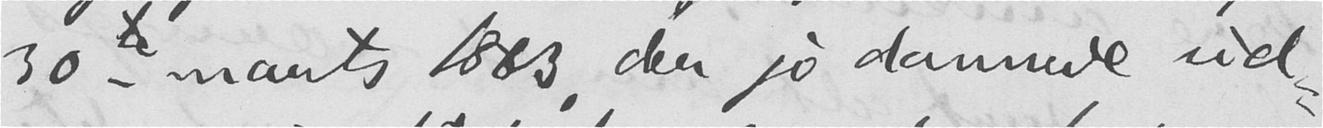30te marts 1883, der jo dannede ud-
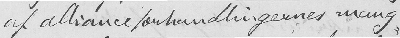af allianceforhandlingernes mang-
